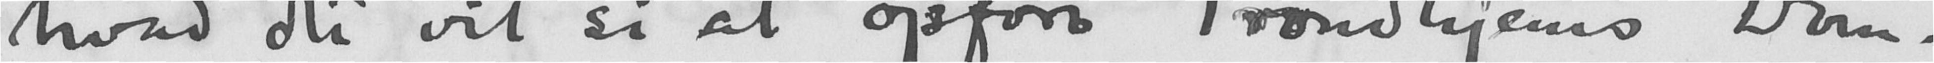hvad det vil si at opføre Trondhjems Dom-
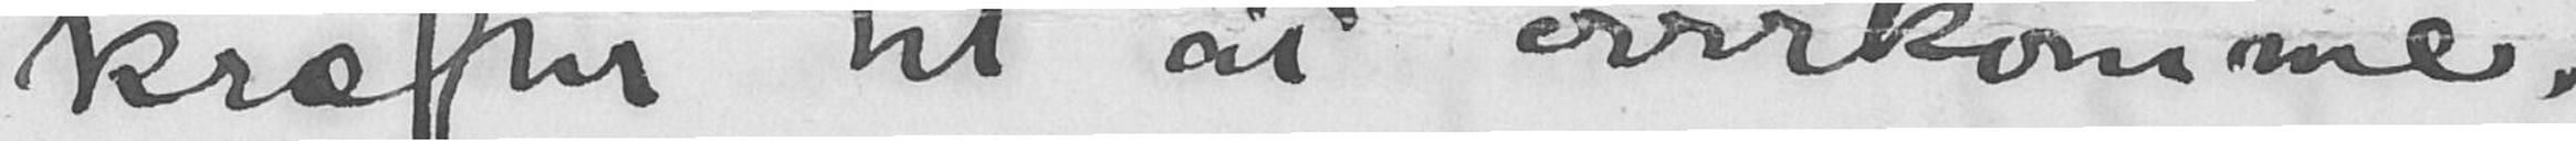kræfter til at overkomme.
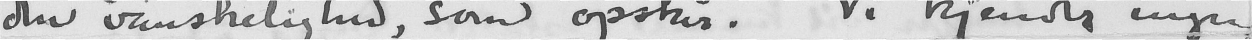den vanskelighed, som opstår. Vi kjender ingen,
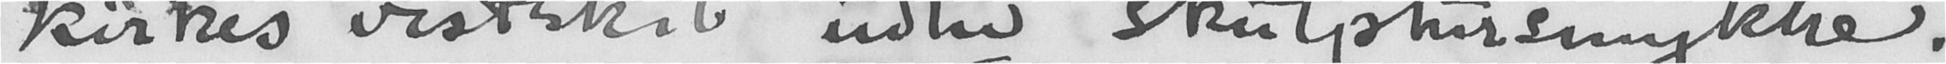kirkes vestskib uden skulptursmykke.
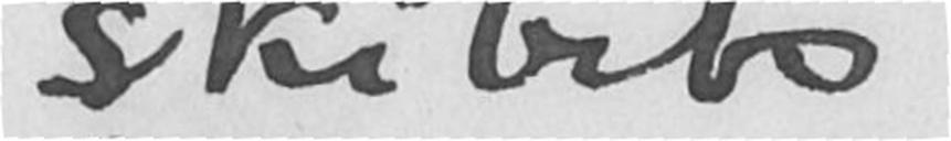skibets
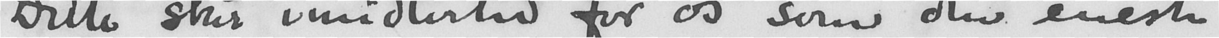Dette står imidlertid for os som den eneste
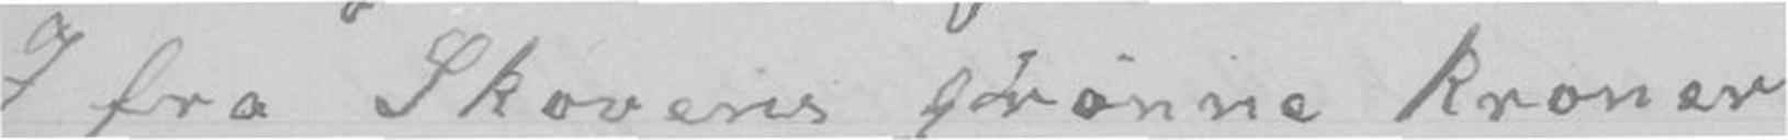I fra Skovens grønne kroner
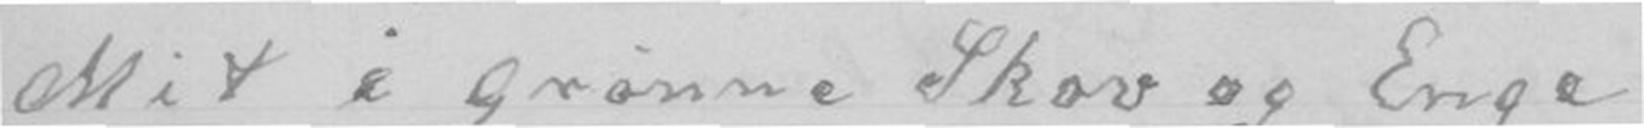Mit i grønne Skov og Enge
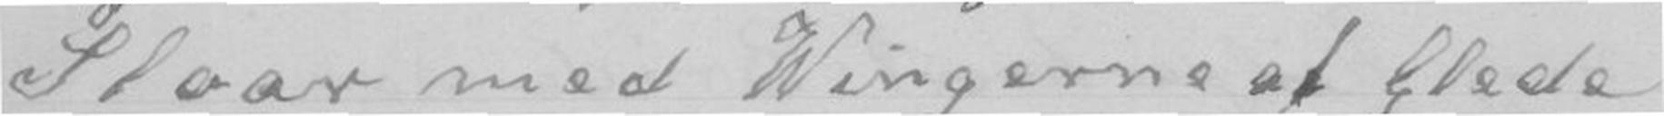Slaar med Wingerne af Glede
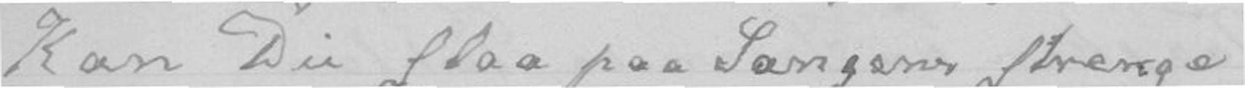Kan Du slaa paa Sangens strenge
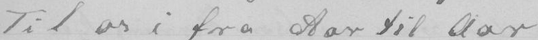Til os i fra Aar til Aar
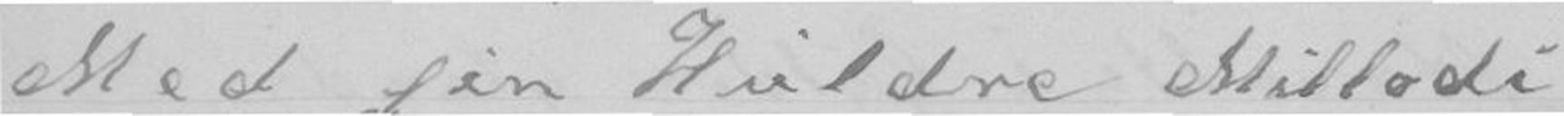Med sin Huldre Mellodi
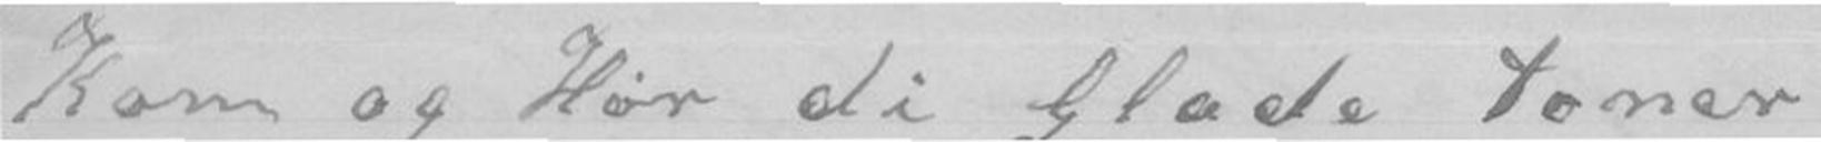Kom og Hør di Glade toner
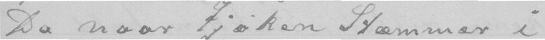Du naar Ijøken Stæmmer i
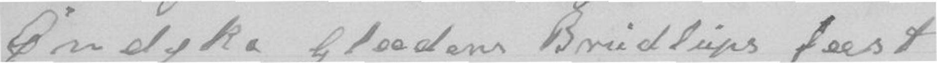Øndske glæden Brudlups fæst
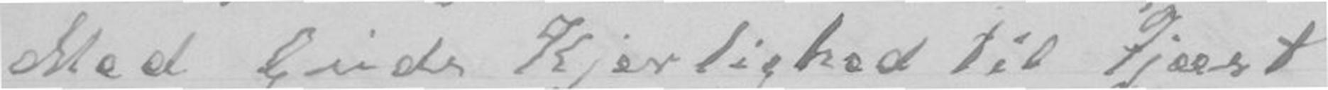Med Guds Kjerlighed til Gjæst
